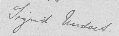Sigrid Undset.
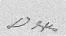Deres
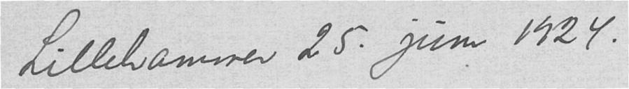Lillehammer 25. jun 1924.
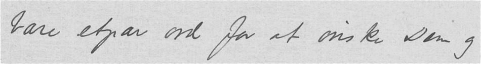bare etpar ord for at ønske Dem og
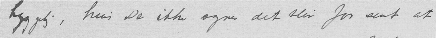hyggelig, hvis De ikke synes det blir for sent at
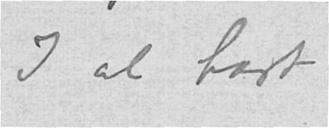I al hast
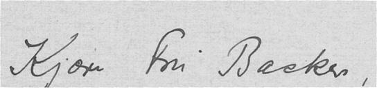Kjære Fru Backer,
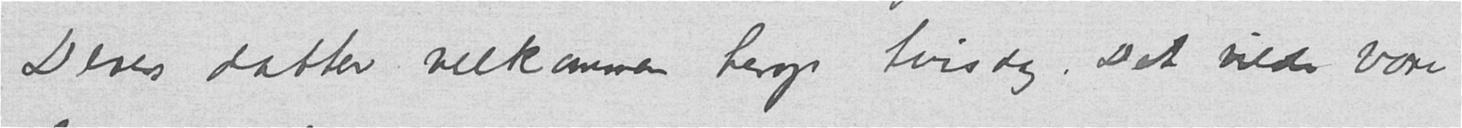Deres datter velkommen herop tirsdag. Det vilde være
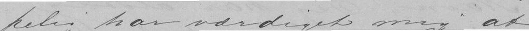kelig har værdiget mig at
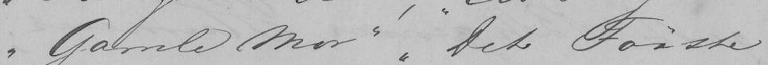Gamle Mor Det Første
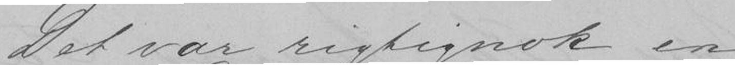Det var rigtignok en
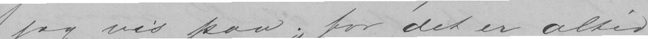jeg vis paa; for det er altid
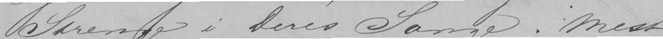Strenge i Deres Sange. Mest
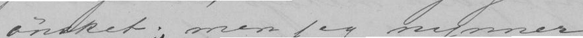ønsket; men jeg nynner
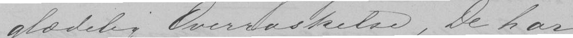glædelig Overraskelse, De har
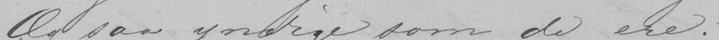Og saa yndige som de ere!
